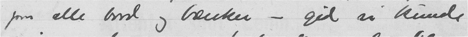paa alle bord og bænker - gid vi kunde
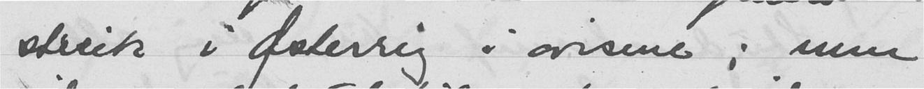streik i Østerrig i avisene; men
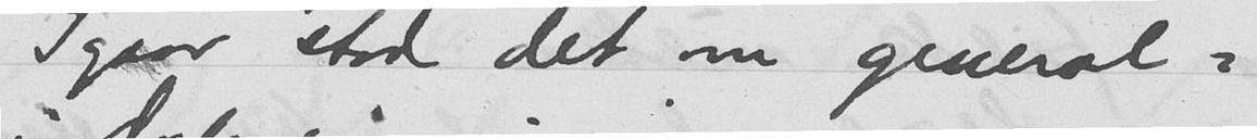Igaar stod det om general-
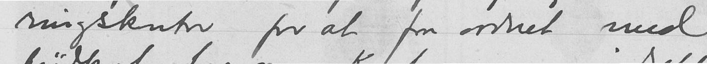ringskontor for at faa ordnet med
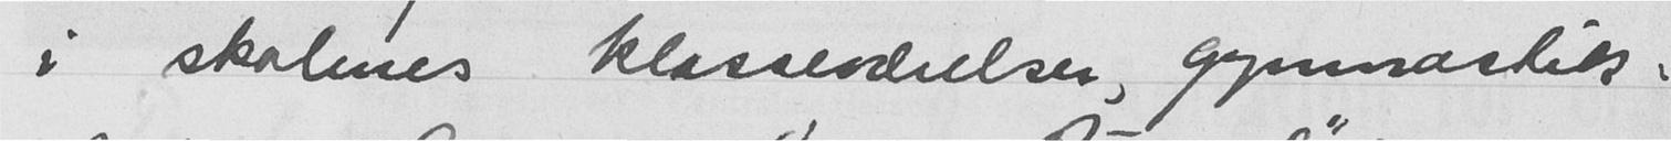i skolenes klasseværelser, gymnastik-
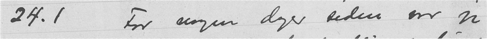24.1 For nogen dager siden var jeg
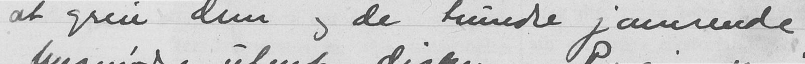at greie dem og de hundre jamrende
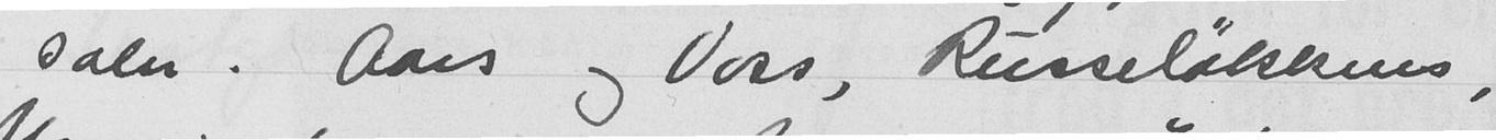saler. Aars og Voss, Russeløkkens,
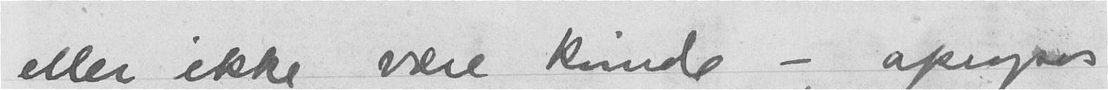eller ikke være kvinde - apropos
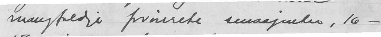mangfoldige forvirrete smaajenter, 16-
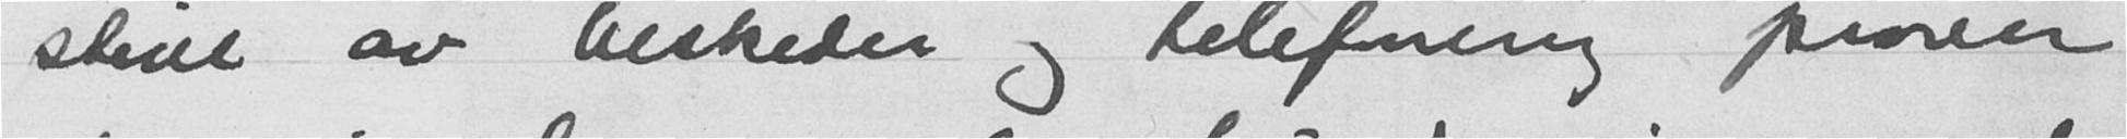stive av beskeder og telefonering prøver
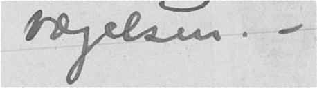vægelsen. -
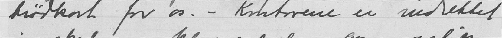brødkort for os. - Kontorene er indrettet
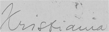Kristiania
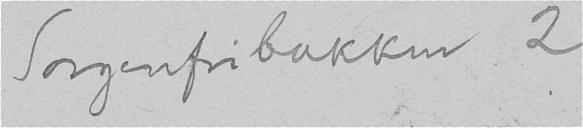Sorgenfribakken 2
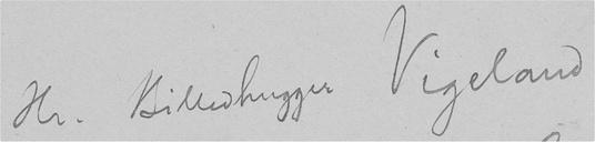Hr. Billedhugger Vigeland
